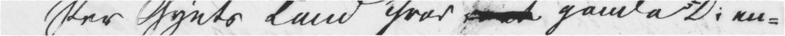Per Gynts Land hvor <gap />t</del> gamle D. en¬
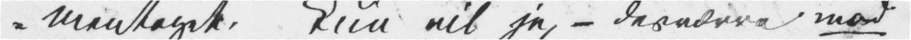mentaget. Kun vil jeg – desværre mod
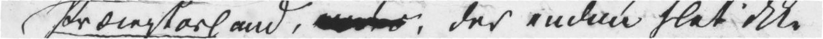Præceptor land, <gap />s</del> der endnu slet ikke
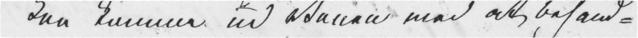kan komme ud Vanen med at behand¬
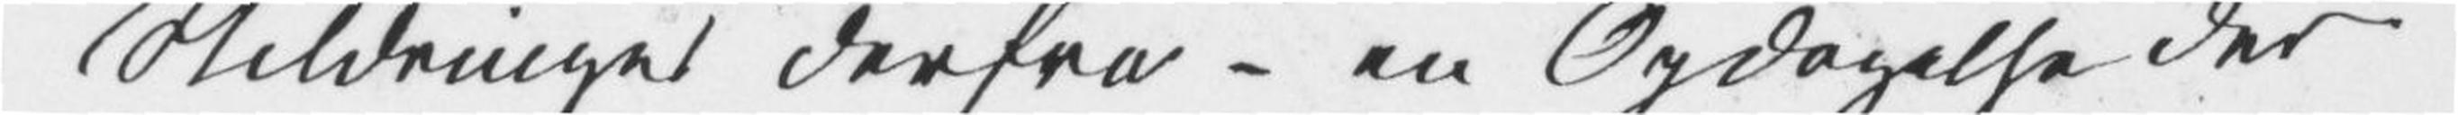Skildringer derfra – en Opdagelse der
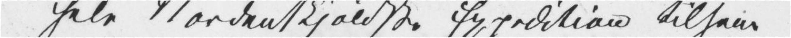hele Nordenskjøldske Expedition tilsam¬
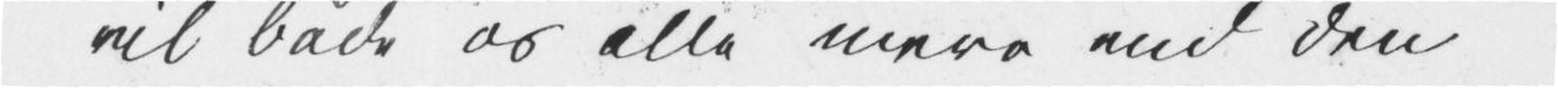vil både os alle mere end den
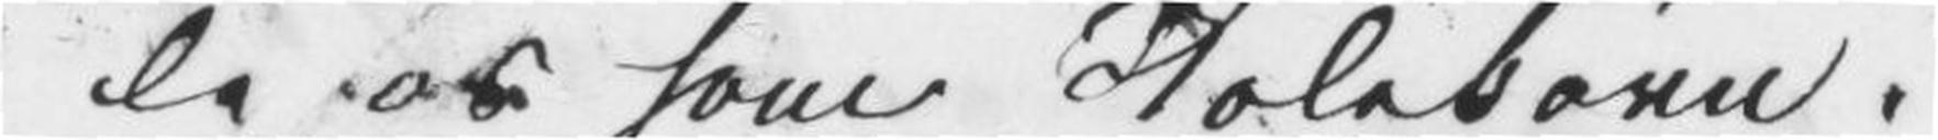le os som Skolebørn.
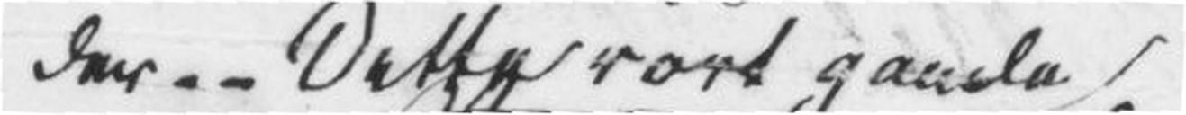der – Dette vort gamle
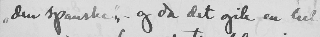"den spanske", - og da det gik en hel
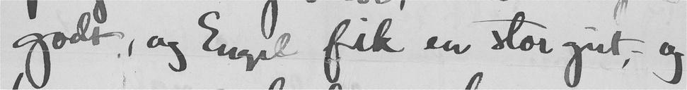godt, og Engel fik en stor gut, - og
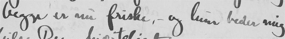begge er nu friske, - og hun beder mig
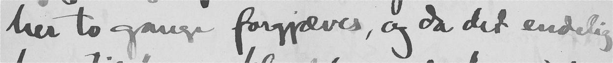her to gange forgjæves, og da det endelig
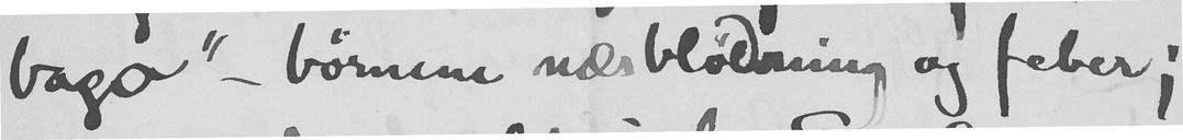bago" - børnene næsblødning og feber;
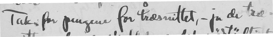Tak for pengene for træsnittet, - ja de træ-
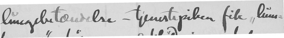lungebetændelse - tjenestepiken fik "lum-
